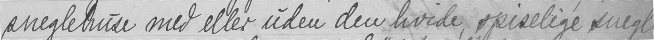sneglehuse med eller uden den hvide, spiselige snegl
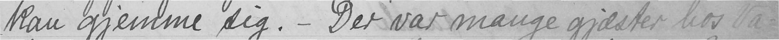kan gjemme sig. - Der var mange gjæster hos Va-
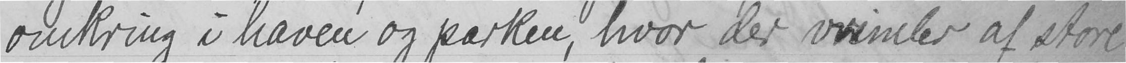omkring i haven og parken, hvor der vrimler af store
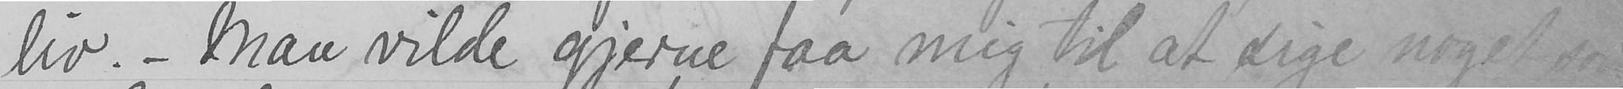liv. - Man vilde gjerne faa mig til at sige noget så
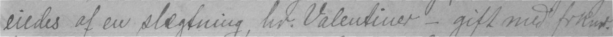eiedes af en slægtning, hr. Valentiner - gift med frknr.
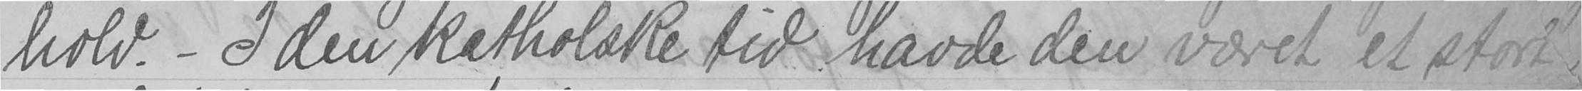hold. - I den katholske tid havde den været et stort,
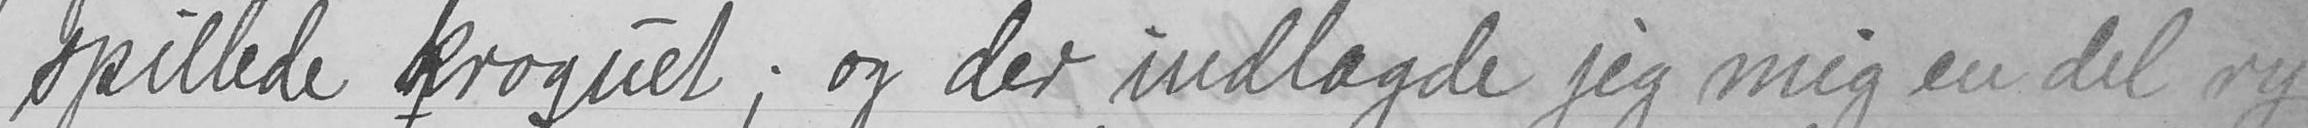spillede kroquet; og der indlagde jeg mig en del ry
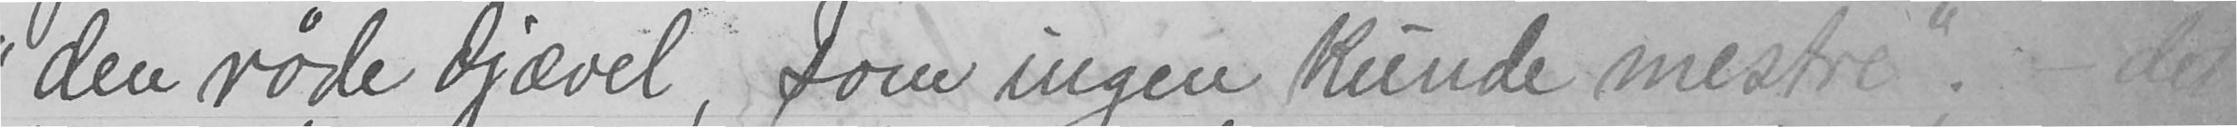"den røde djævel, som ingen kunde mestre"; - det
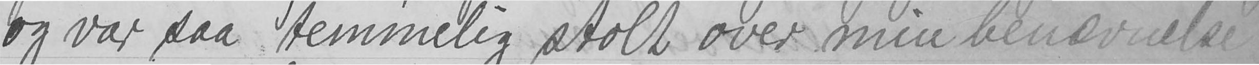og var saa temmelig stolt over min benævnelse
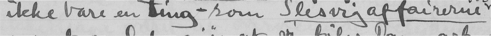ikke bare en ting - som Slesvig affairerne
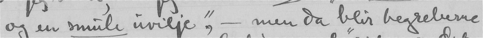og en smule uvilje", - men da blir begreberne
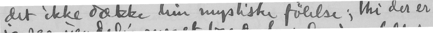det ikke dække hin mystiske følelse; thi der er
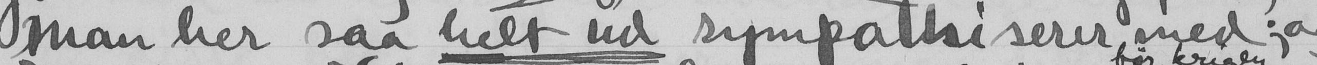man her saa helt ud sympathiserer med; og
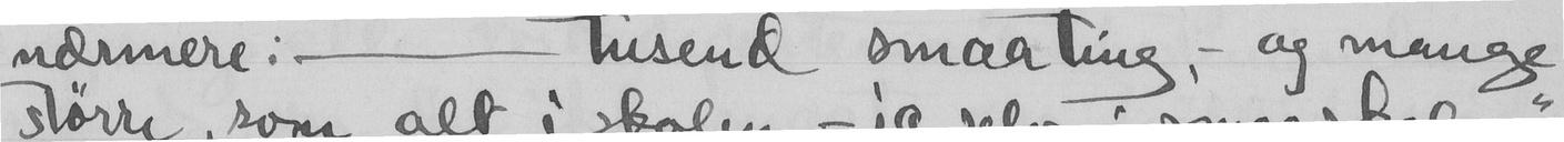nærmere: - tusend smaating, - og mange
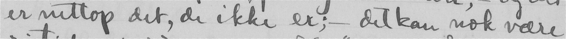er nettop det, de ikke er; - det kan nok være
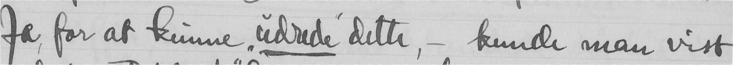Ja, for at kunne "udrede" dette, - kunde man vist
